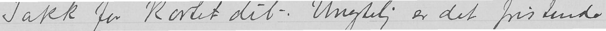Takk for kortet dit. Unegtelig er det fristende
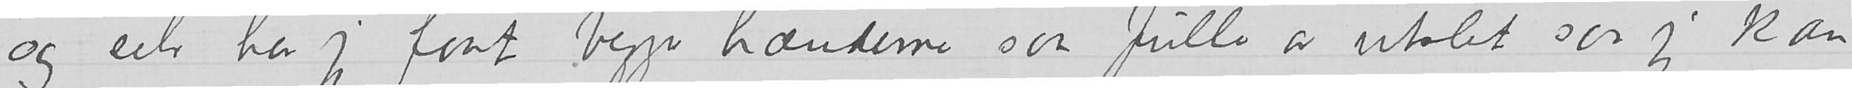og selv har jeg faat begge hænderne saa fulle av utslet saa jeg kan
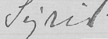Sigrid.
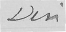Din
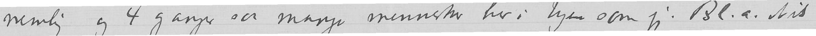nemlig og 4 ganger saa mange mennesker her i byen som jeg. Bl.a. Ais
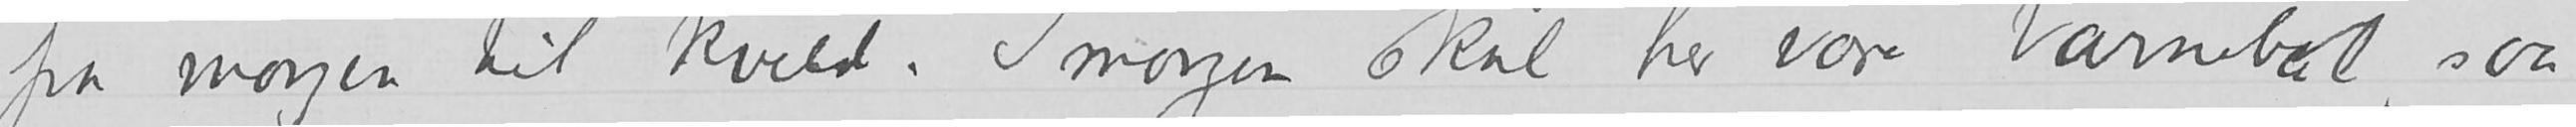fra morgen til kveld. Imorgen skal her være barnebal, saa
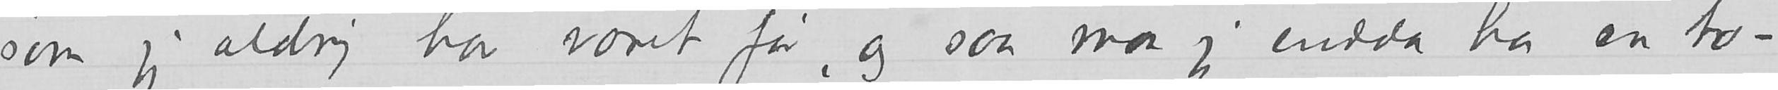som jeg aldrig har været før, og saa må jeg endda ha en to-
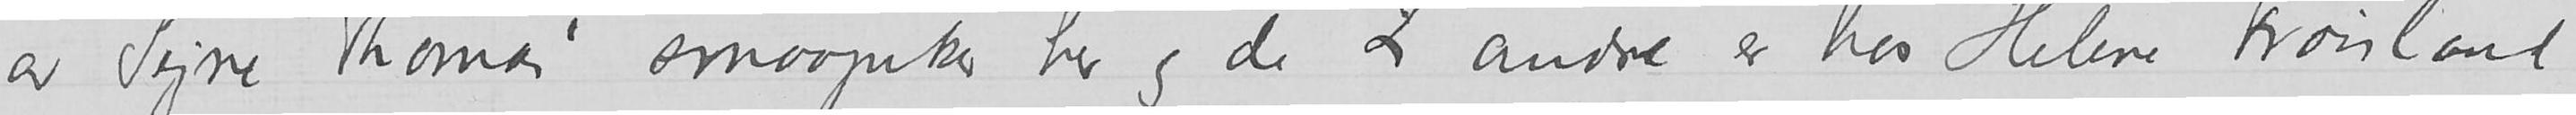av Signe Thomas’ smaapiker her og de 2 andre er hos Helene Frøisland
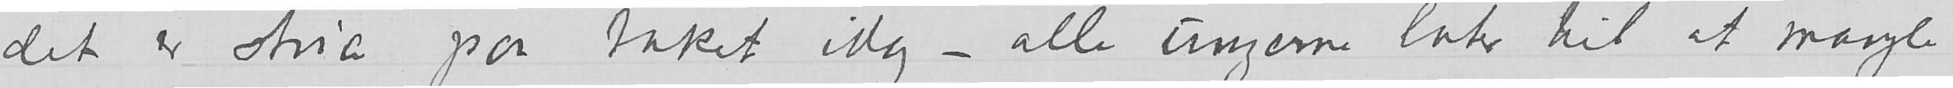det er stua paa taket idag – alle ungerne later til at mangle
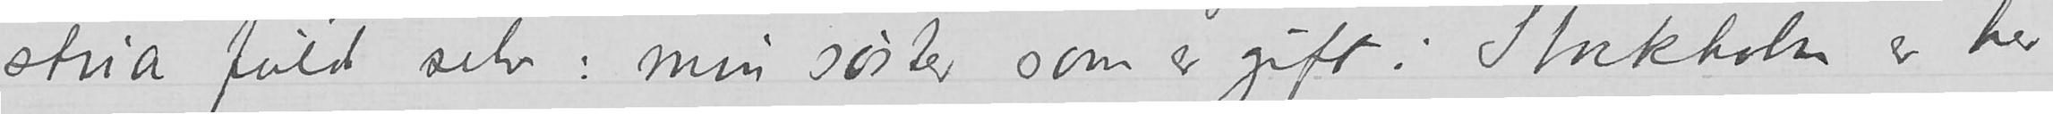stua fuld selv: min søster som er gift i Stokholm er her
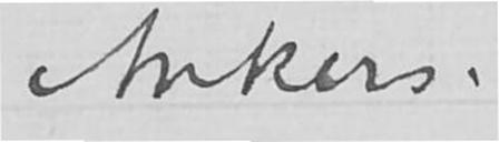Ankers.
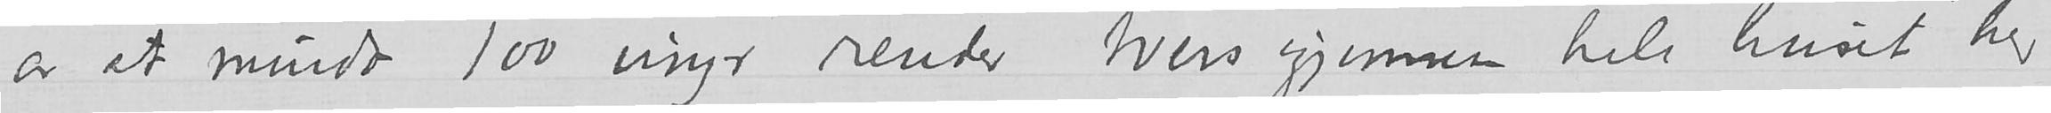av at mindst 100 unger render tvers gjennem hele huset her
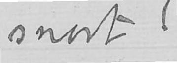snart!
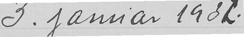3. januar 1932.
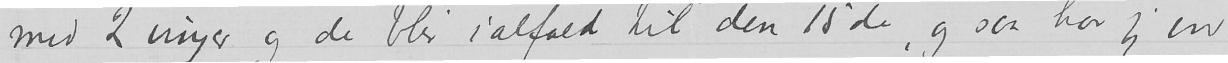med 2 unger og de blir ialfald til den 15de, og saa har jeg en
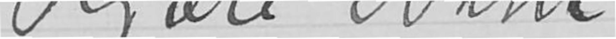Kjære Nini,
